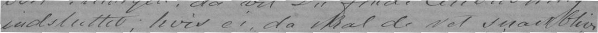indsluttet; hvis ei, da skal de ret snart blive
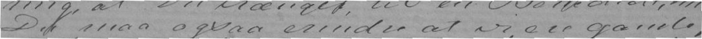Du maa ogsaa erindre at vi ere gamle,
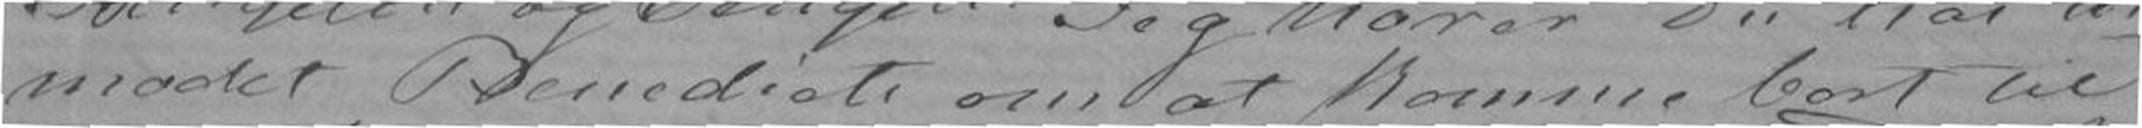modet Benedicte om at komme bort til
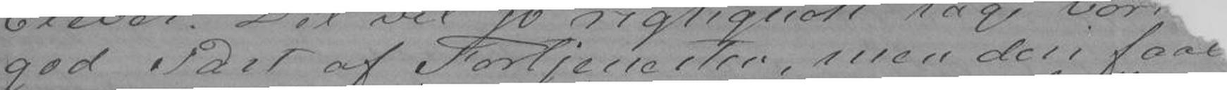god Part af Fortjenesten, men deri faa
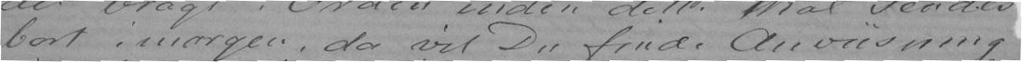bort imorgen, da vil Du finde Anvisning
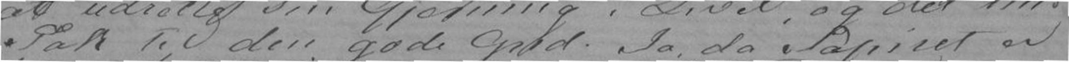Tak til den gode Gud. Ja, da Papiret er
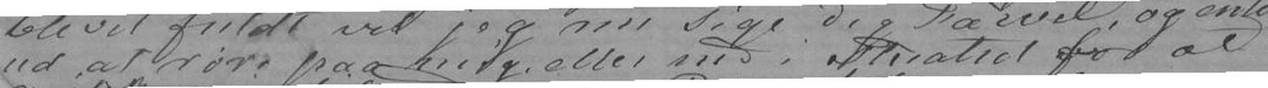ud at røre paa mig eller ned i Theatret for at
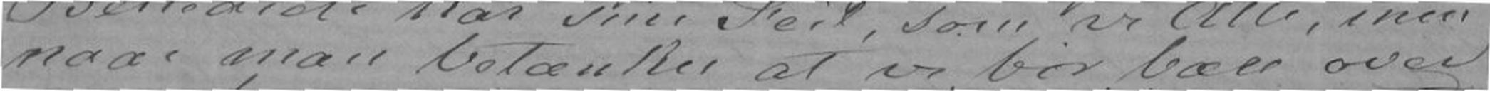naar man betænker at vi bør bære over
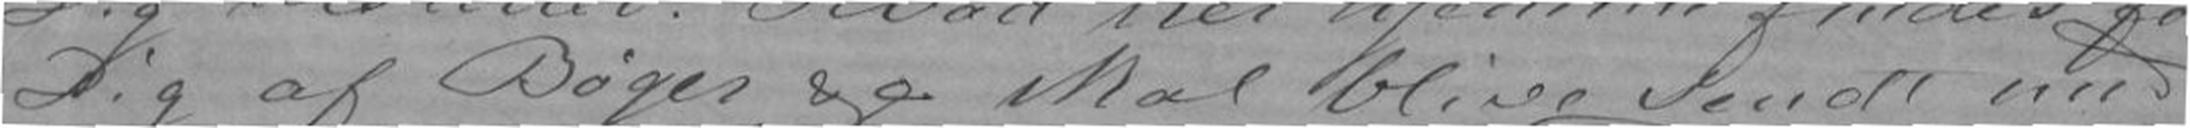Dig af Bøger exc skal blive sendt med
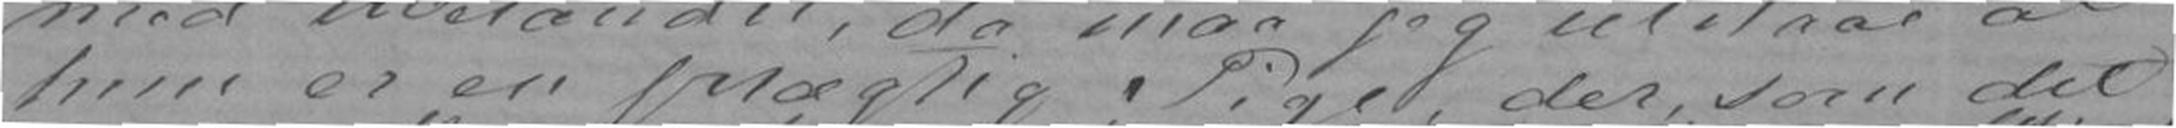hun er en prægtig Pige, der, som det
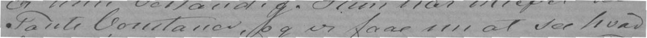Tante Constance, og vi faar nu at see hvad
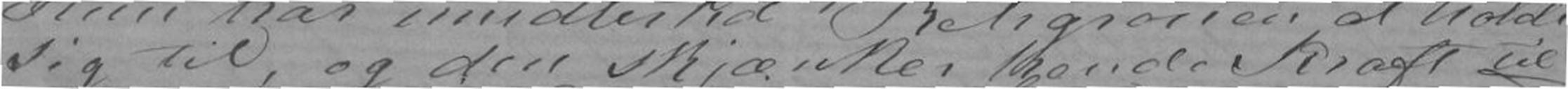sig til, og den skjænker hende Kraft til
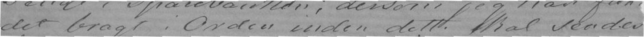det bragt i Orden inden dette skal sendes
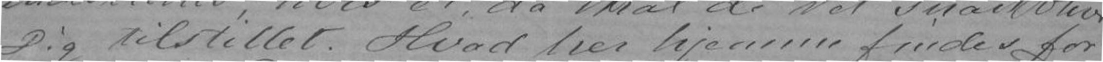Dig tilstillet. Hvad her hjemme findes for
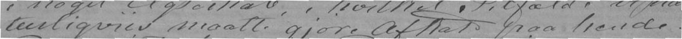turligviis maatte gjøre Afkald paa hende.
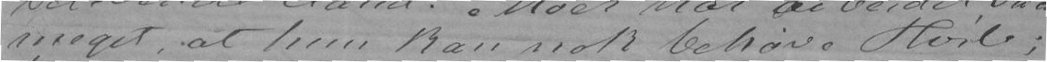meget, at hun kan nok behøve Hvile;
